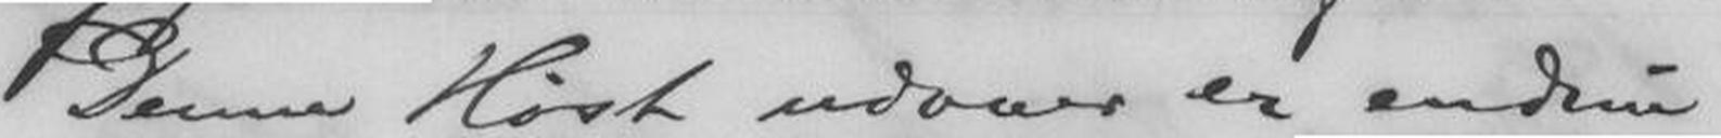Denne Høst udover er endnu
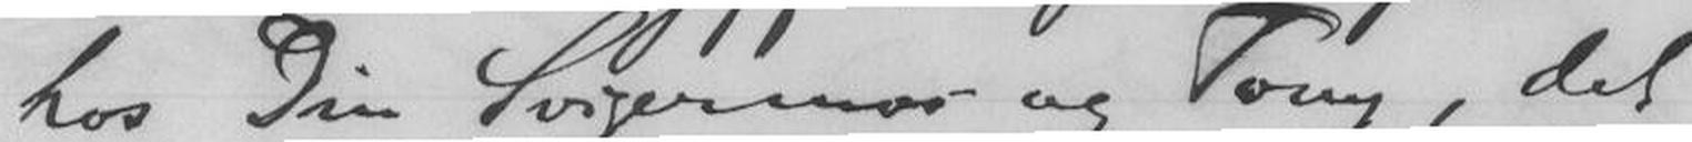hos Din Svigermor og Tony, det
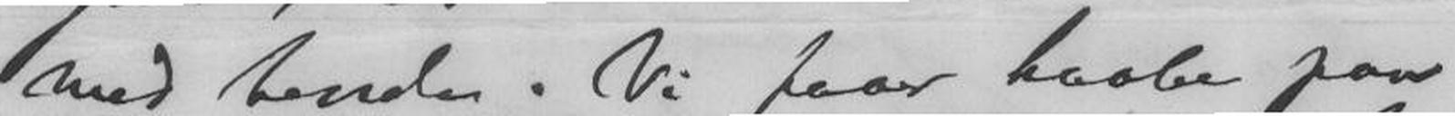med hende. Vi faar haaabe paa
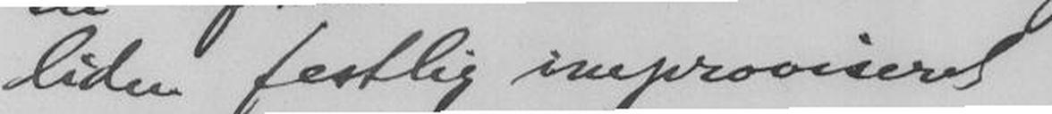liden festlig improviseret Tilstelning.
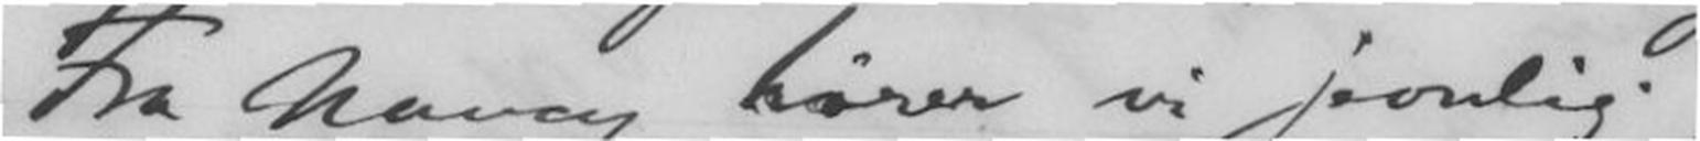Fra Nancy hører vi jevnlig.
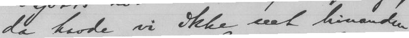da havde vi ikke seet hinanden
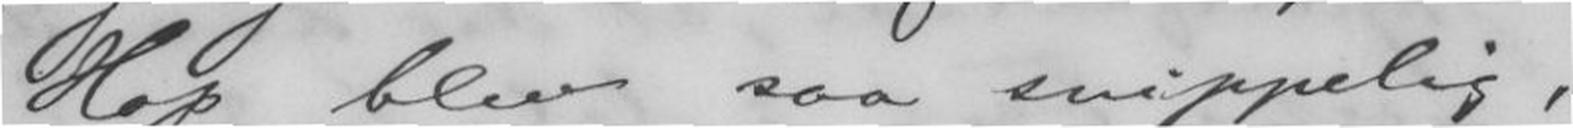Hop blev saa svippelig,
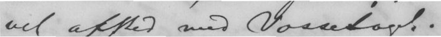vet afsted med Vossetoget.
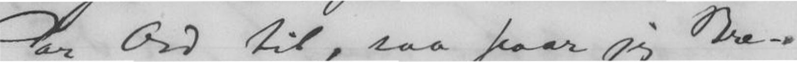Par Ord til, saa faar jeg Bre-
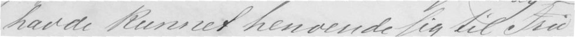havde kunnet henvende sig til Fru
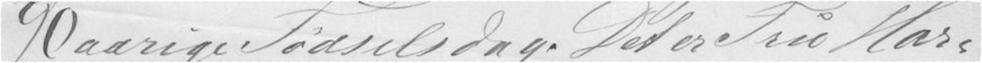90 aarige Fødselsdag. Det er Fru Hare
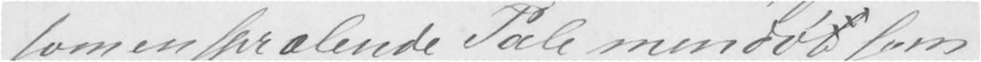som en sprælende Tale men som
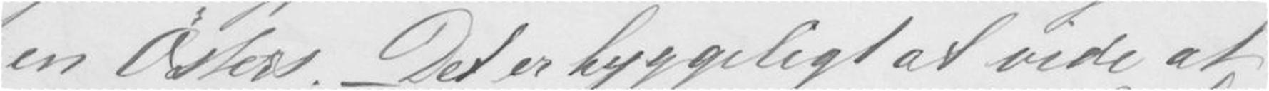en sters.- Det er hyggelegt at vide at
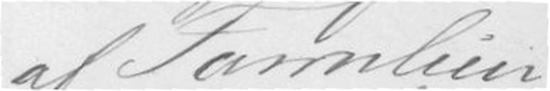af Familien
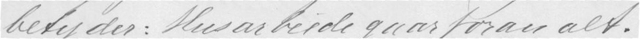betyder: Husarbeide gaar foran alt.
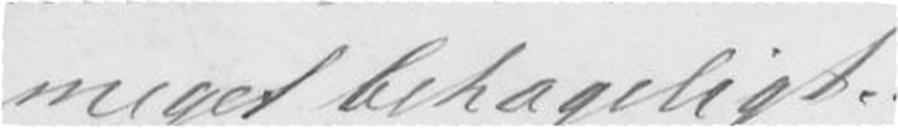meget behageligt.
